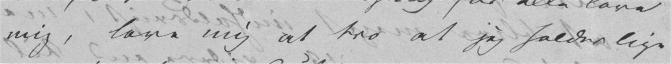mig, love mig at tro at jeg holder lige
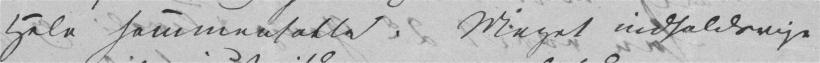Hele sammensatte. Meget indholdsrige
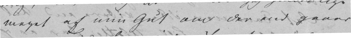meget af min Gut om der end gaaer
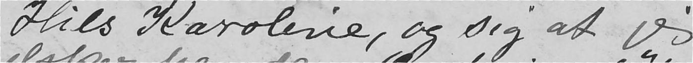Hils Karoline, og sig at jeg
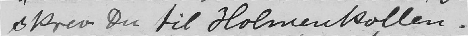skrev Du til Holmenkollen.
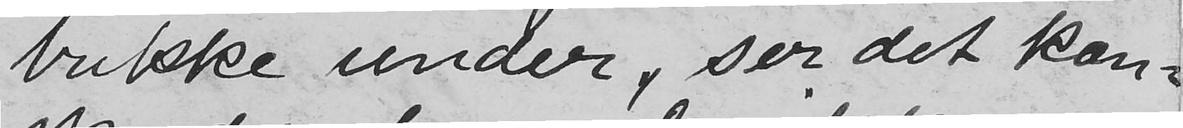bukke under, ser det kan-
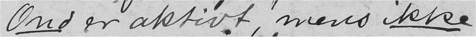Onder aktivt, mens ikke
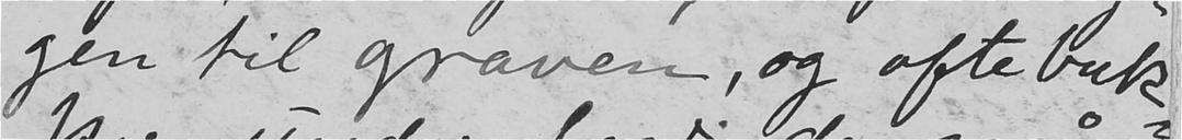gen til graven, og ofte buk-
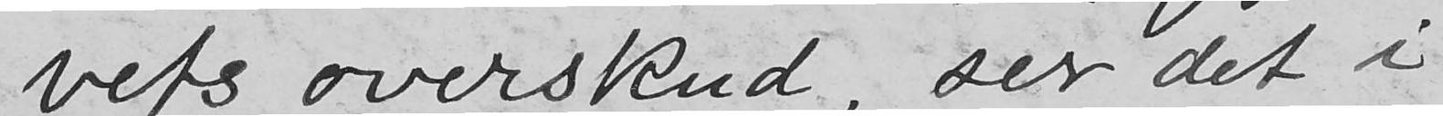vets overskud, ser det i
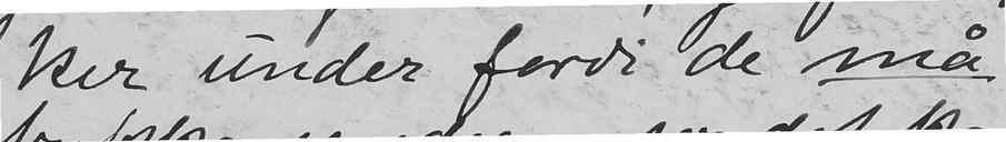ker under fordi de må
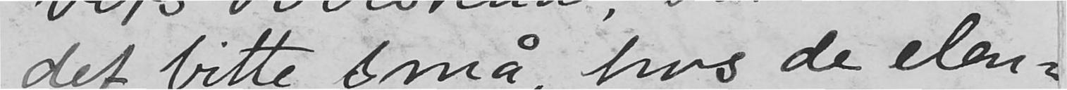det bitte små hvis de elen-
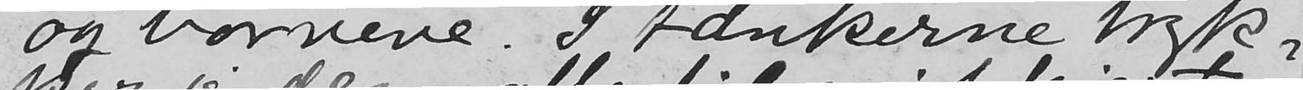og barnene. I tankerne tryk-
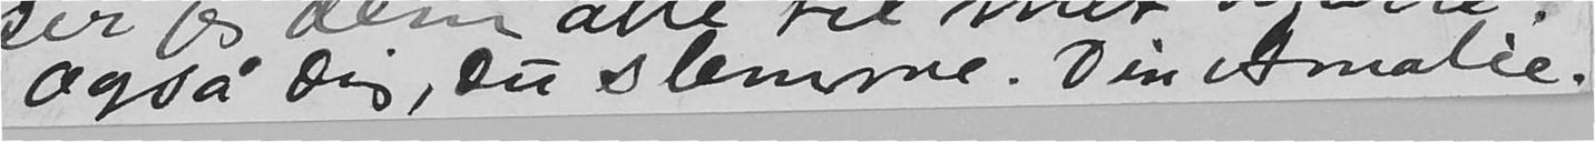Også Dig, Du slemme. Din Amalie.
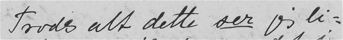Trods alt dette ser jeg li-
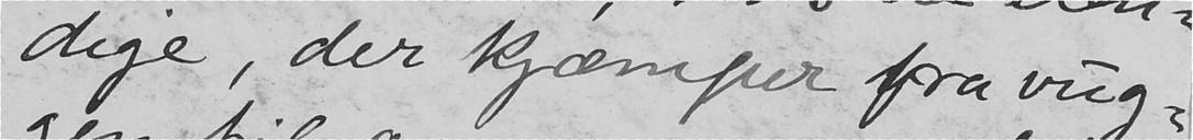dige, der kjæmper fra vug-
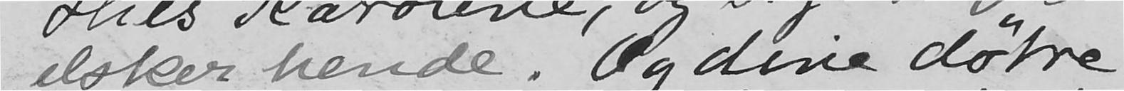elsker hende. Og dine døtre
